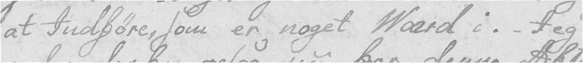at Indføre, som er noget Værd i. – Jeg
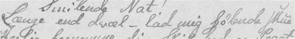Længe end dvæl – lad mig følende skue
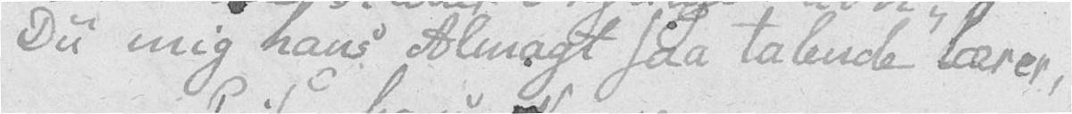Du mig hans Almagt saa talende lærer,
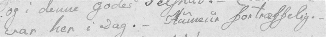var her i Dag. – Humeur fortræffelig. –
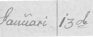Januari 13de
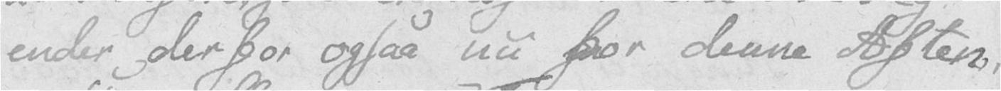ender derfor ogsaa nu for denne Aften,
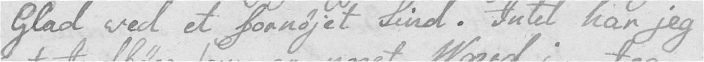Glad ved et fornøjet Sind. Intet har jeg
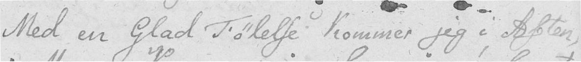Med en Glad Følelse kommer jeg i Aften,
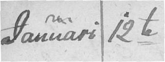Januari 12te
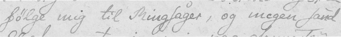følge mig til Ringsager, og megen sand
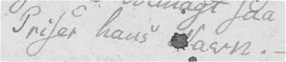Priser hans Navn. –
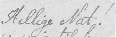Hellige Nat!
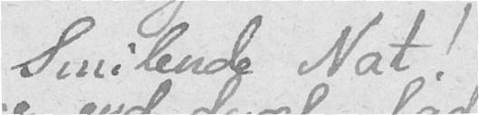Smilende Nat!
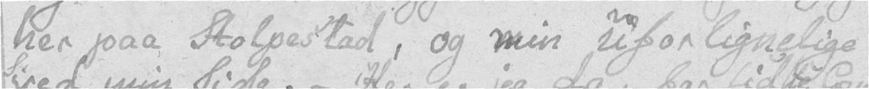her paa Stolpestad, og min Uforlignelige
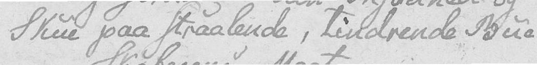Skue paa straalende, tindrende Bue
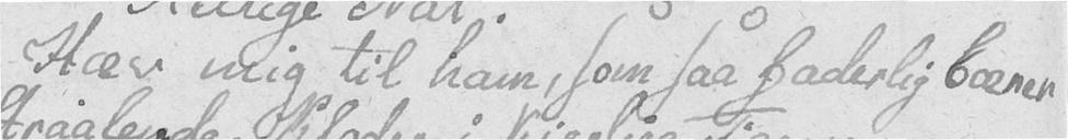Hæv mig til ham, som saa faderlig bærer
